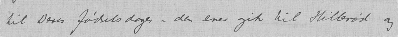til Deres fødselsdager - den ene gik til Hillerød og
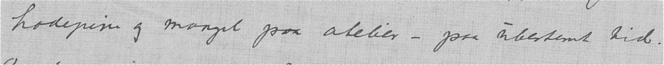hodepine og mangel paa atelier – paa ubestemt tid.
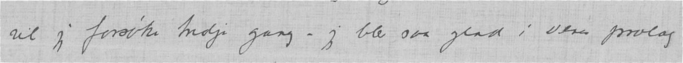vil jeg forsøke tredje gang – jeg blev saa glad i Deres prolog
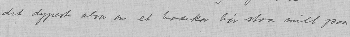det dypeste alvor om et badekar bør staa mitt paa
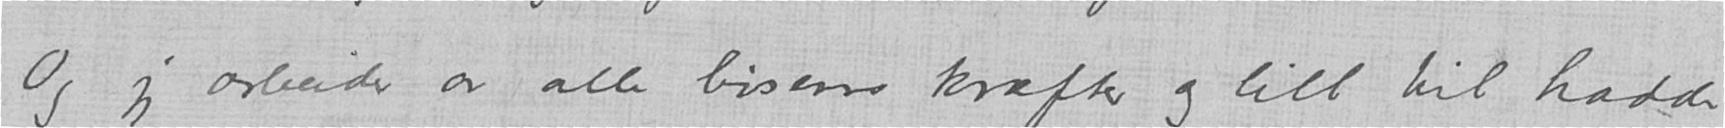Og jeg arbeider av alle livsens kræfter og litt til hadde
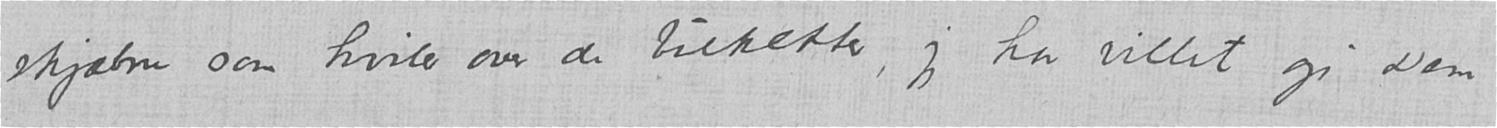skjæbne som hviler over de buketter, jeg har villet gi Dem
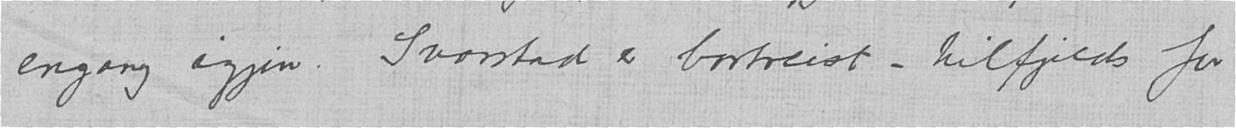engang igjen. Svarstad er bortreist - tilfjelds for
